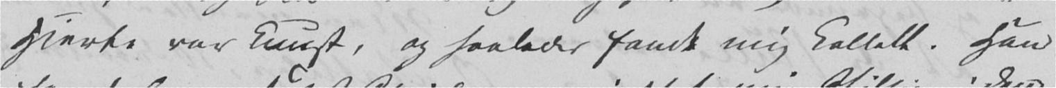Hjerte var knust, og saaledes fandt mig Collett. Han
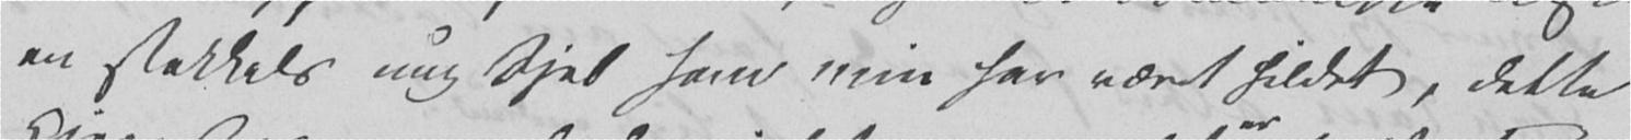en stakkels ung Sjel som min har været hildet, dette
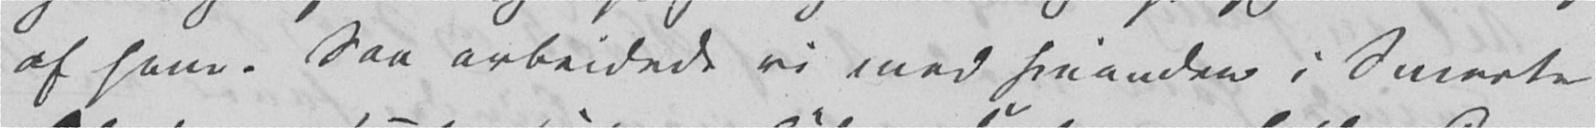af ham. Saa arbeidede vi med hinanden i Smerte
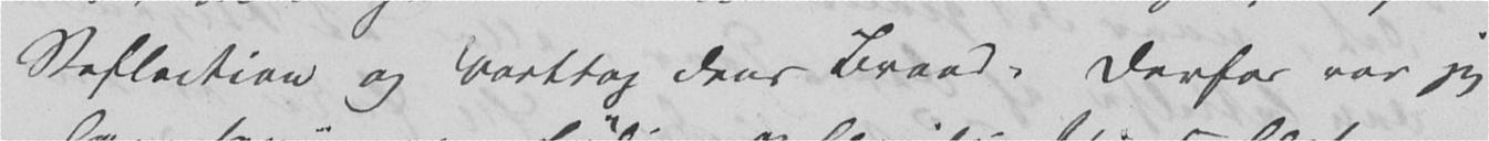Reflection og borttog dens Braad. Derfor var jeg
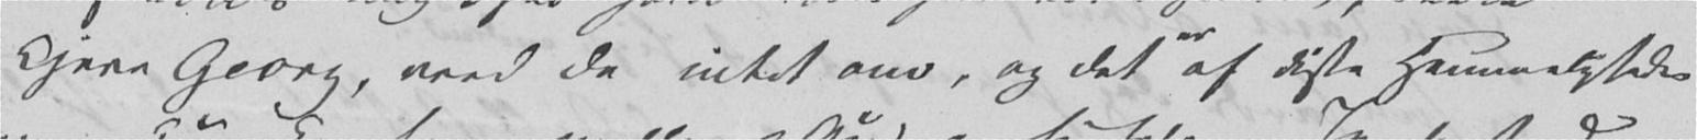kjere Georg, veed De intet om, og det er af disse Hemmeligheder
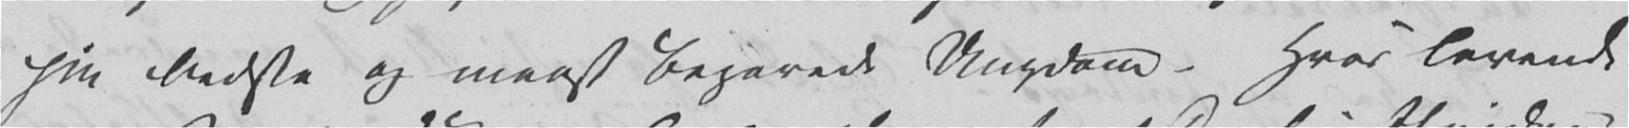sin bedste og meest begavede Ungdom. Hvor levende
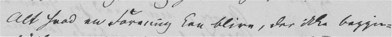Alt hvad en Forening kan blive, der ikke begyn-
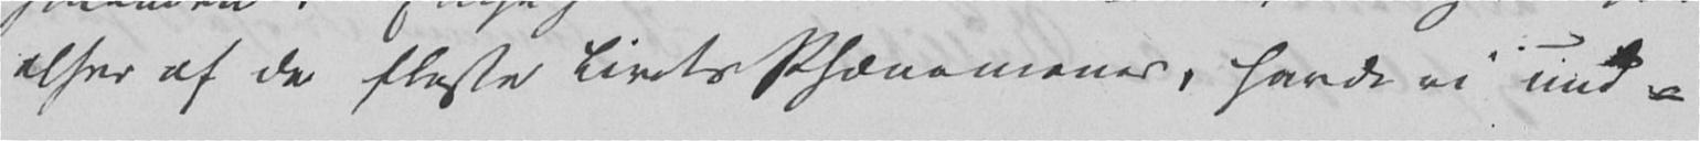elser af de fleste Livets Phænomener, havde vi uud-
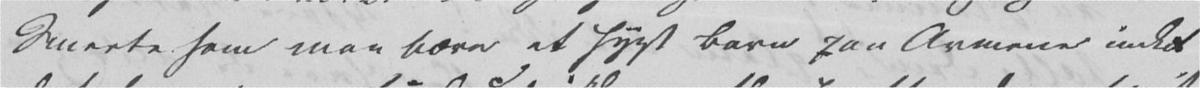Smerte som man bærer et sygt Barn paa Armene indtil
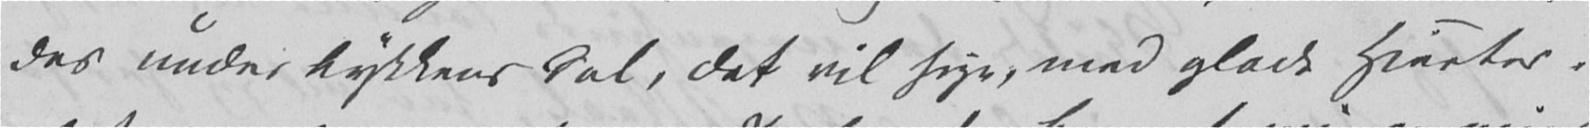des under Lykkens Sol, det vil sige, med glade Hjerter,
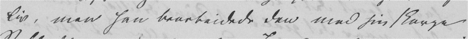Liv, men han bearbeidede den med sin skarpe
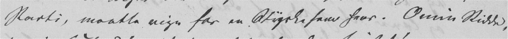Parti, maatte vige for en Styrke som hans. O min Ridder,
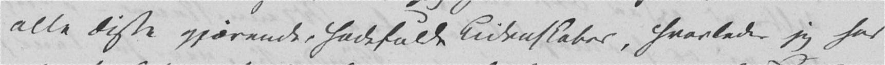alle disse gjærende, hadefulde Lidenskaber, hvorledes jeg har
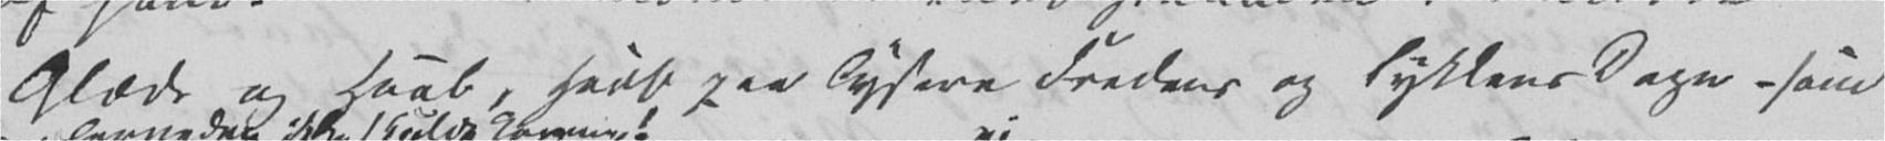Glæde og Haab, Haab paa lysere Fredens og Lykkens Dage – som
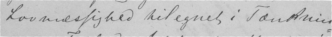Lovmæssighed tilegnet i Tænknin-
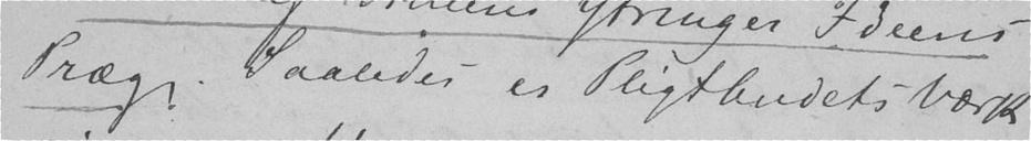Præg. Saaledes er Pligtbudets Værk
